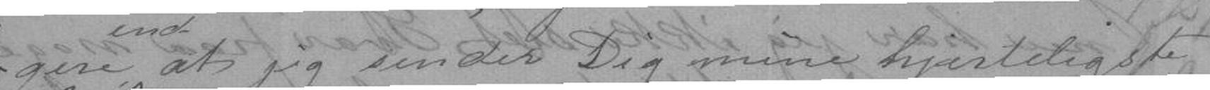gen at jeg under Dig mine hjerteligste
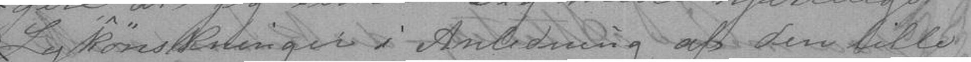Lykønskninger i Anledning af den lille
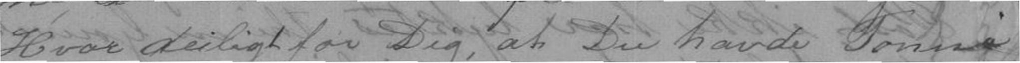Hvor deiligt for Dig at Du havde Tonny
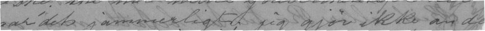gaar det jammerligt; jeg gjør ikke ande
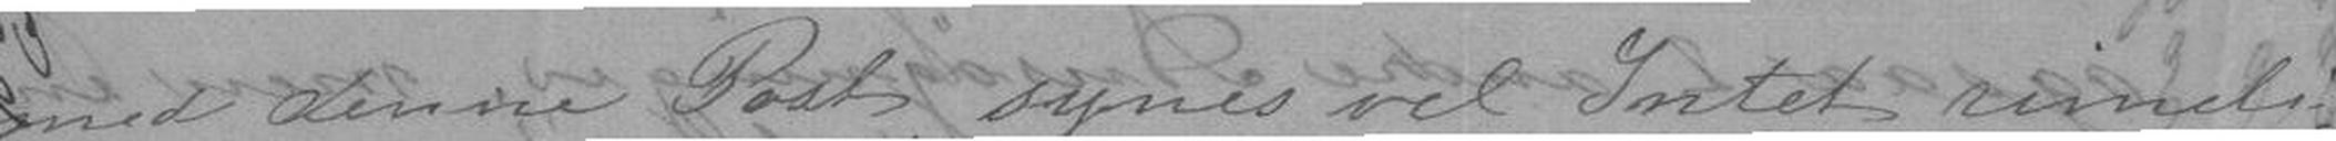med denne Post, synes vel Intet rimeli-
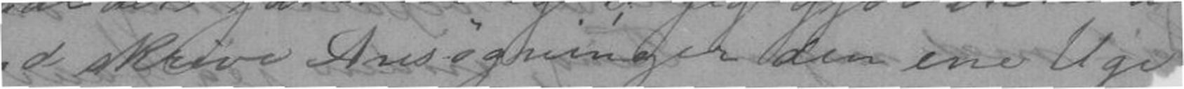end skrive Ansøgninger den ene Uge
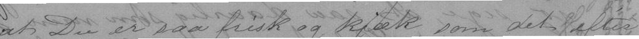at Du er saa frisk og Kjæk, som det efter
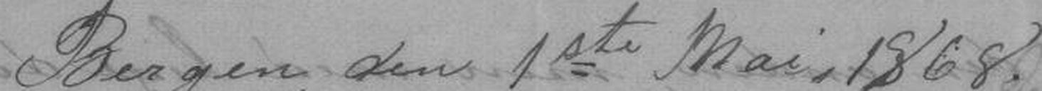Bergen, den 1ste Mai 1868.
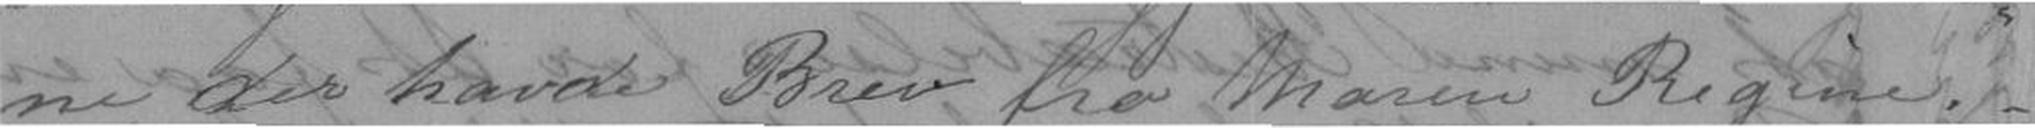ne, der havde Brev fra Maren Regine.
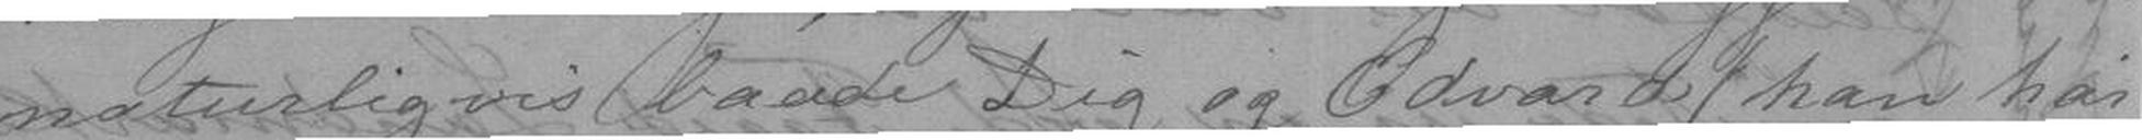naturligvis baade Dig og Edvard (han har
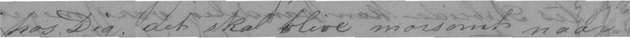hos Dig; det skal blive morsomt naar
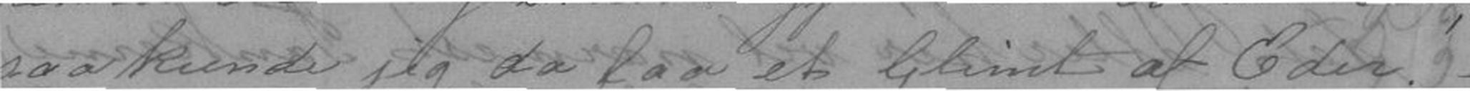saa kunde jeg da faa et Glimt af Eder. -
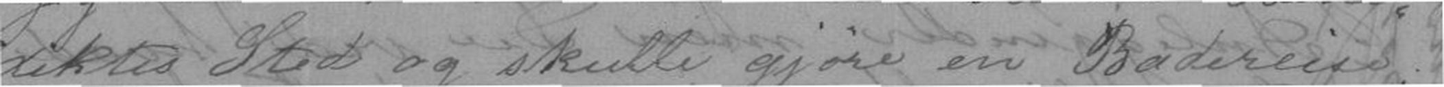diktes Sted og skulle gjøre en Badereise,
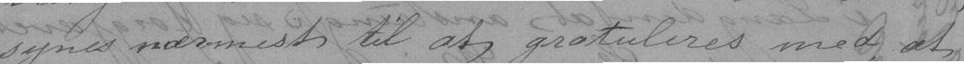synes nærmest til at gratuleres med at
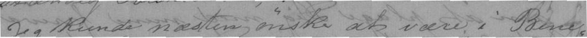Jeg kunde næsten ønske at være i Bene-
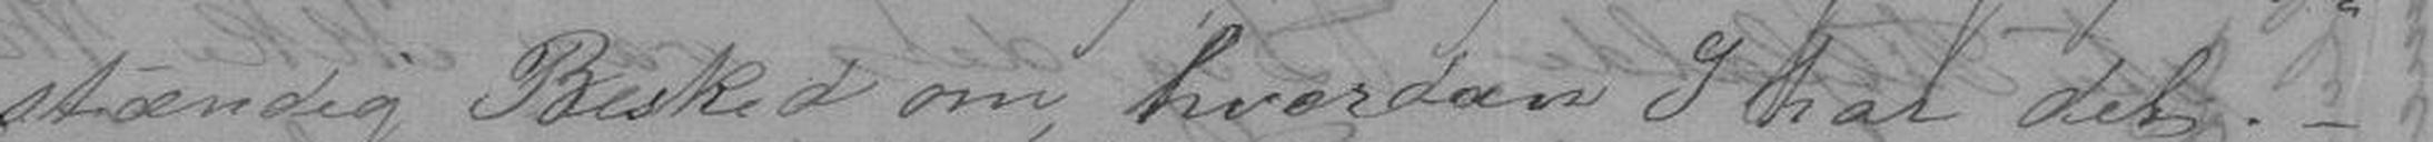stændig Besked om, hvordan I har det.
